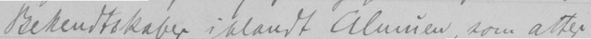Bekendskaber iblandt Almuen, som atter
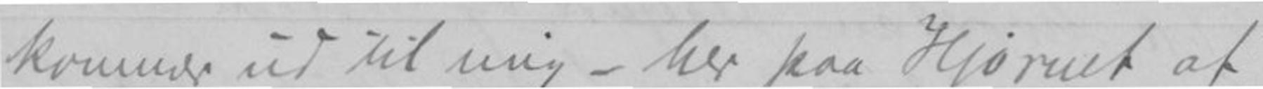kommer ud til mig - her paa Hjørnet af
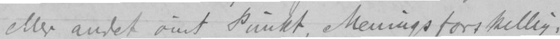eller andet ømt Punkt, Meningsforskellig
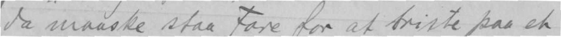da maaske staa Fare for at briste paa et
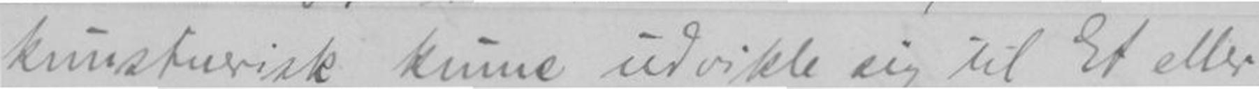kunstneriske kunne udvikle sig til Et eller
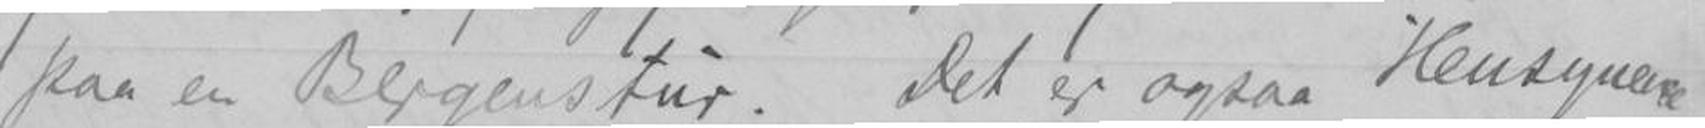paa en Bergenstur. Det er ogsaa ikke Hensynene
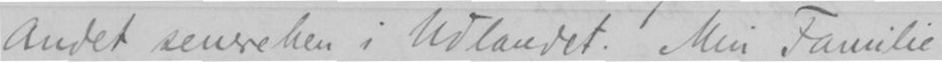Andet senere hen i Udlandet. Min Familie
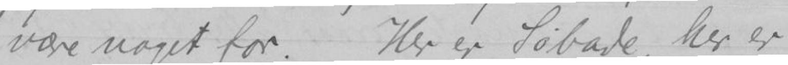være noget for. Her er Søbade, her er
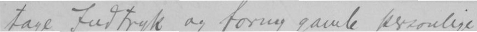tage Indtryk og fornye gamle personlige
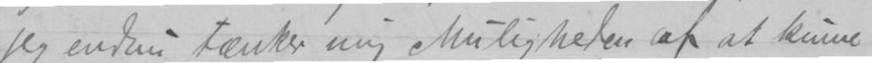jeg endnu tænker mig Muligheden af at kunne
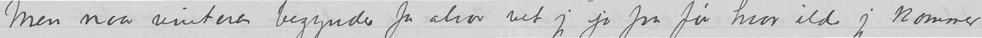Men naar vinteren begynder for alvor vet jeg jo fra før hvor ilde jeg kommer
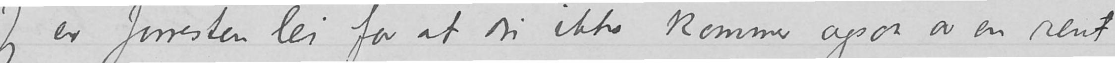Jeg er forresten lei for at du ikke kommer opover av en rent
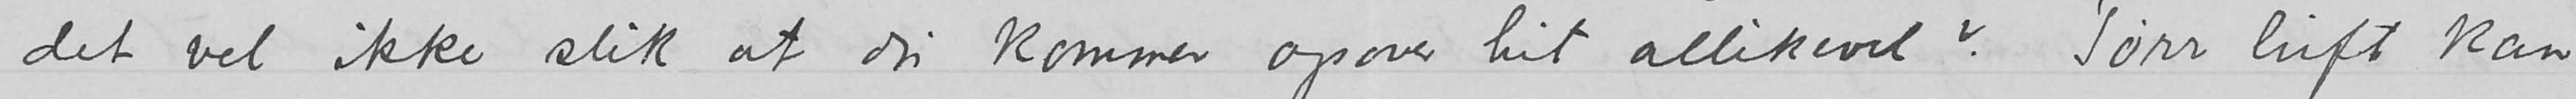det vel ikke slik at du kommer opover hit allikevel?Tørr luft kan
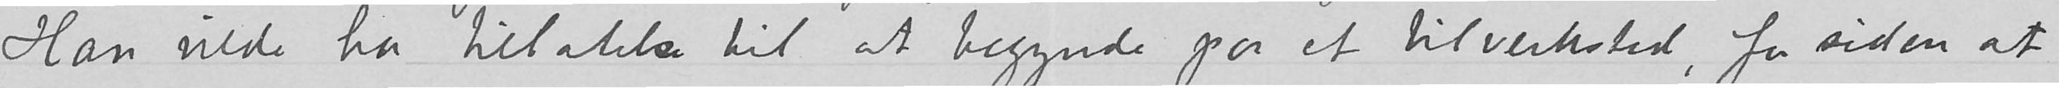Han vilde ha tillatelse til at begynde paa et bilverksted,for siden at
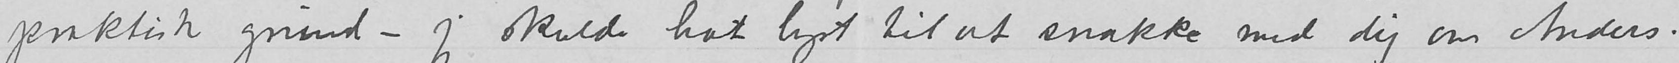praktisk grund – jeg skulde hat lyst til at snakke med dig om Anders.
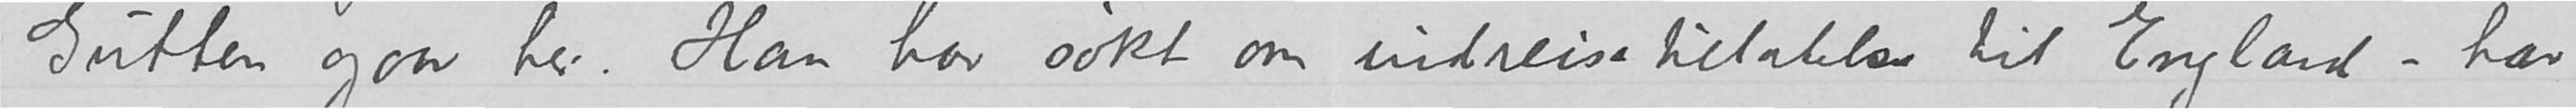Gutten gaar her.  Han har søkt om indreisetillatelse til England – har
